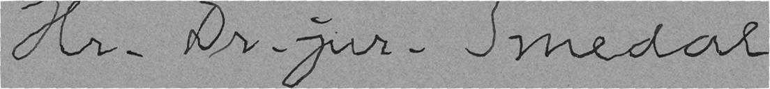Hr. Dr. jur. Smedal
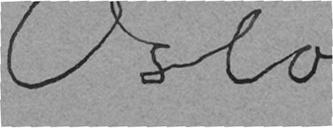Oslo
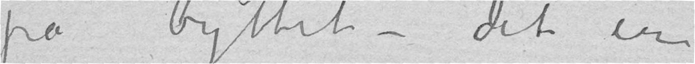på byttet – det er
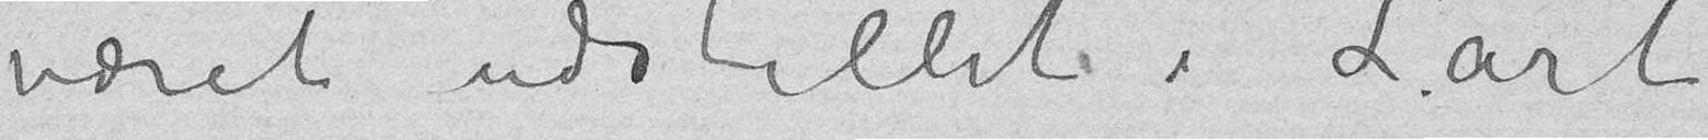været udstillet i L’art
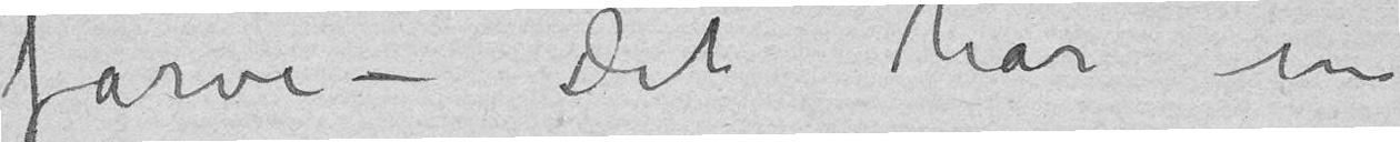farve – Det har nu
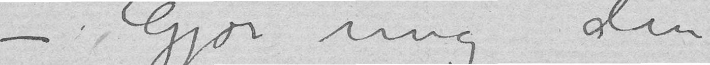– Gjør mig den
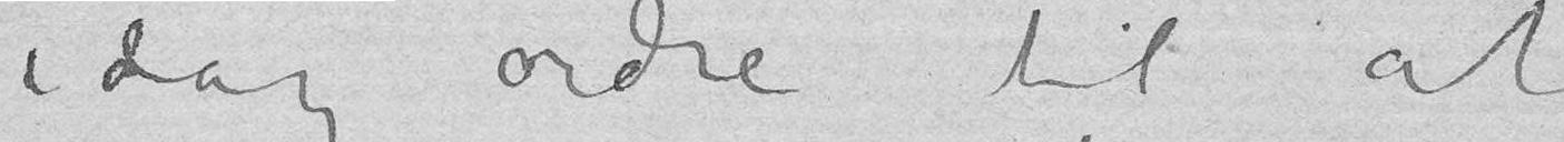i dag ordre til at
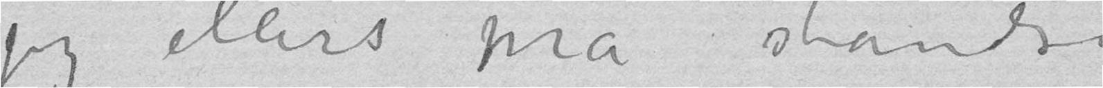jeg ellers må standse
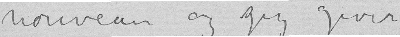nouveau og jeg giver
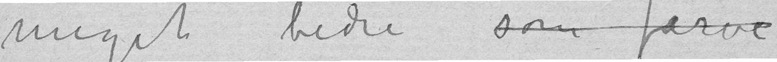meget bedre som farve
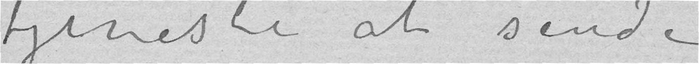tjeneste at sende
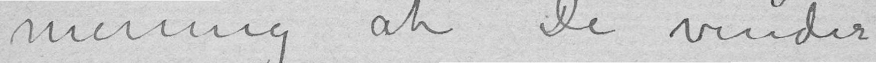mening at De vinder
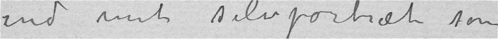end mit selvportræt som
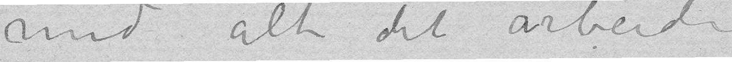med alt det arbeide
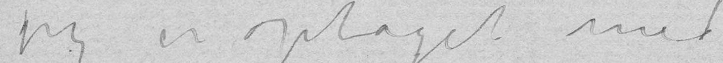jeg er optaget med
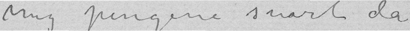mig pengene snart da
